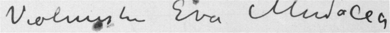Violinistin Eva Mudocci
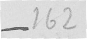162
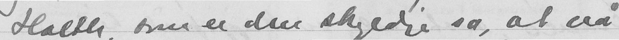Holth, som er den skyldige sa, at nå
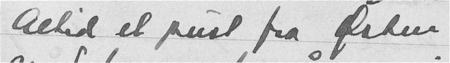Altid et pust fra Østen
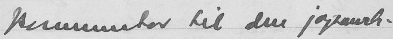kommentar til den japansk-
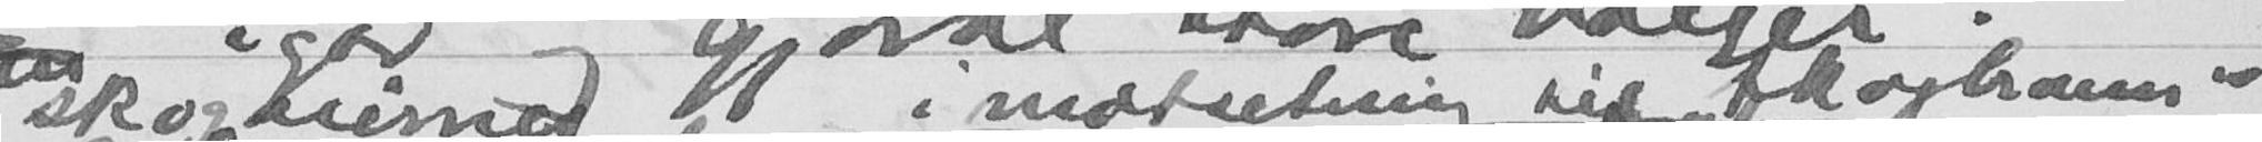skokserne i motsetning til "skogbrann"
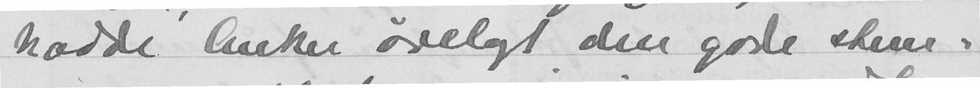hadde Anker ødelagt den gode stem-
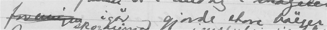igår og gjorde store bølger
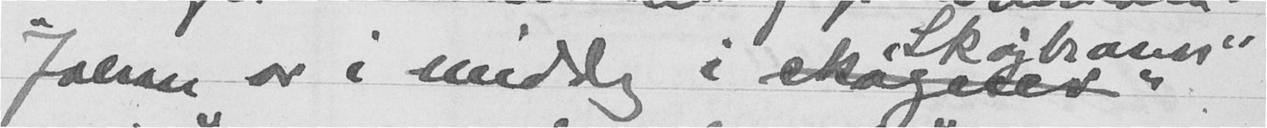Johan var i middag i  "Skøihaven"
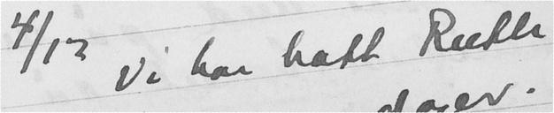4/12 Vi har hatt Ruth
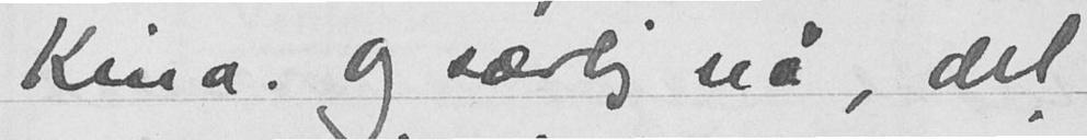Kina. Og særlig nå, det
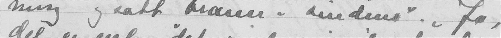ning og satt brann i sindene". "Ja,
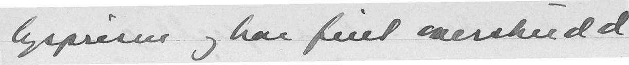lysprisen og har, fint overskudd
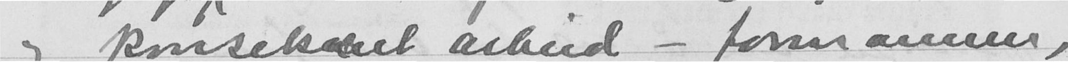og konsekvent arbeid - formannen,
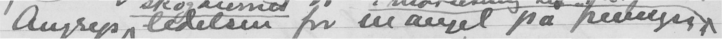Angrep på ledelsen for mangel på penger
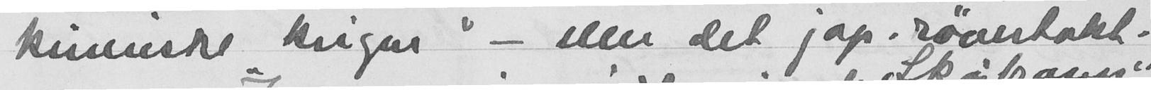kinesiske "krigen" - eller det jap. røvertokt.
